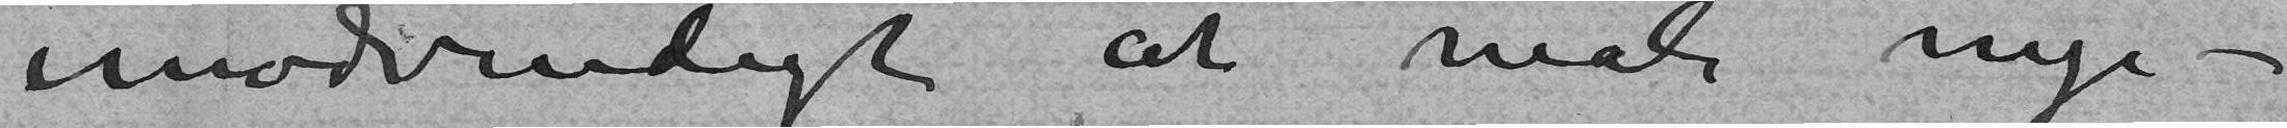unødvendigt at male nye –
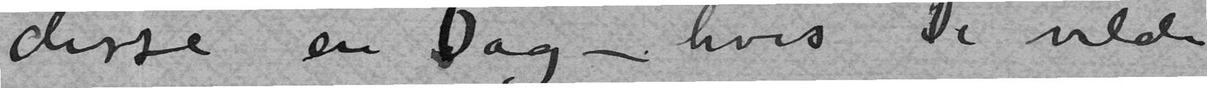disse en Dag – hvis De vilde
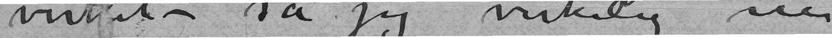virket – så jeg virkelig nu
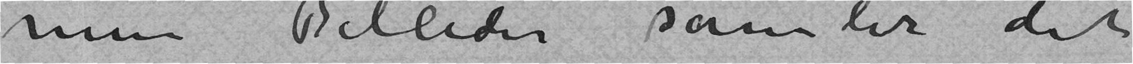mine Billeder samler det
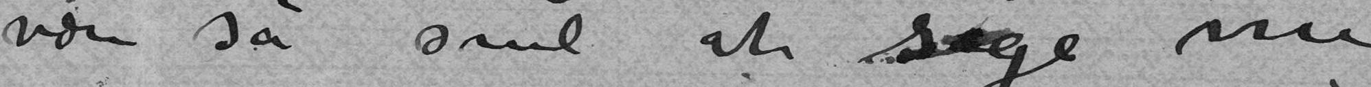være så snil at sige mig
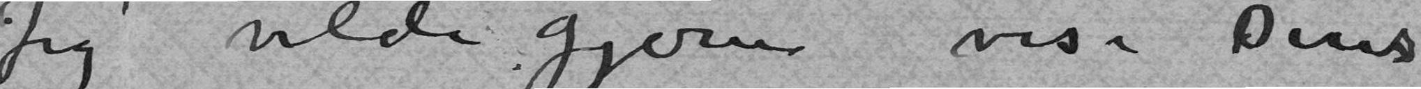Jeg vilde gjerne vise Dem
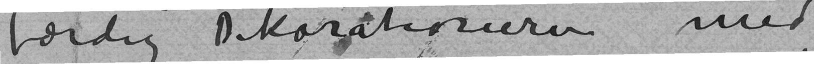færdig Dekorationerne med
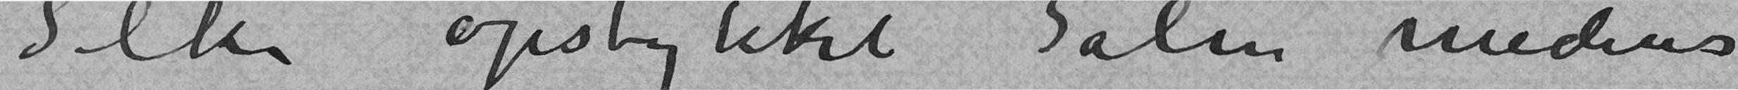Silke opstykket Salen medens
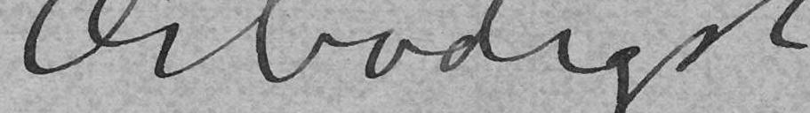Ærbødigst
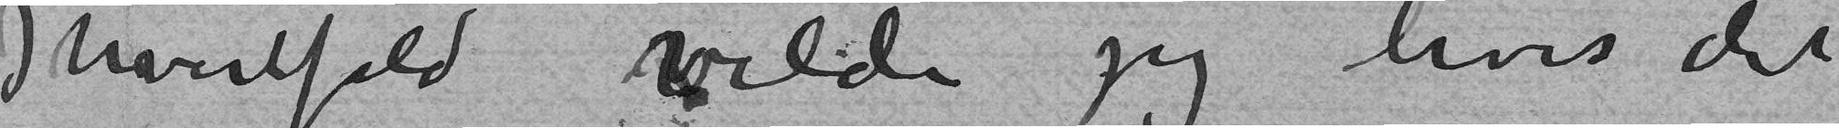Ihvertfald vilde jeg hvis der
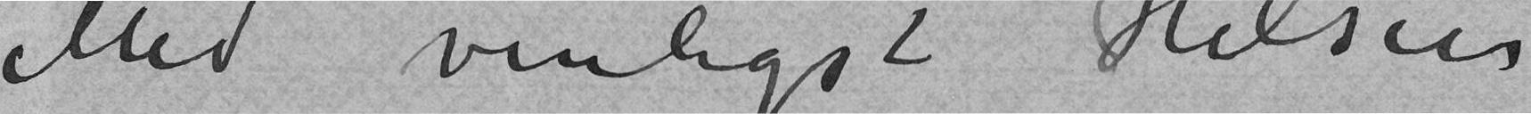Med venligst Hilsen
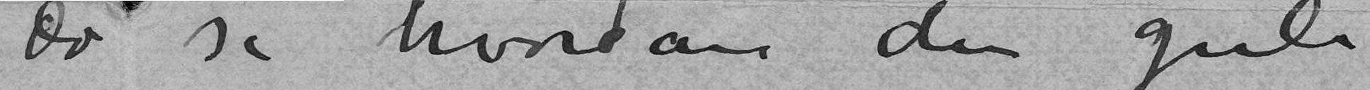da se hvordan den gule
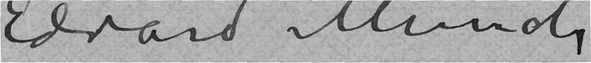Edvard Munch
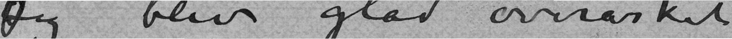Jeg blev glad overasket
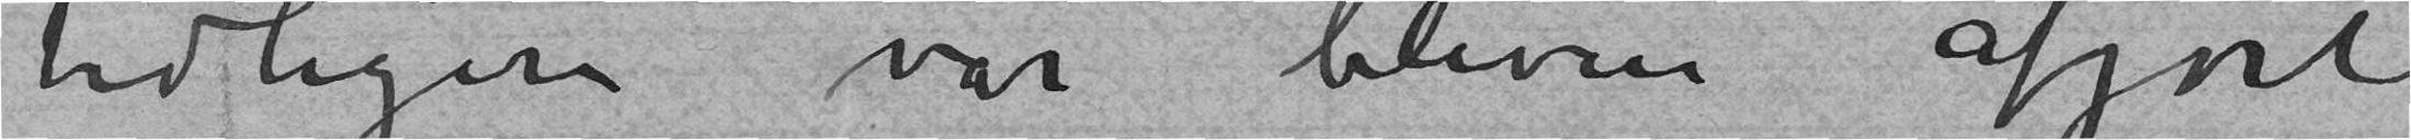tidligere var bleven afgjort
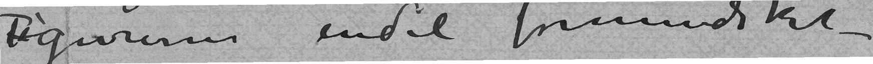Figurerne endel formindsket –
